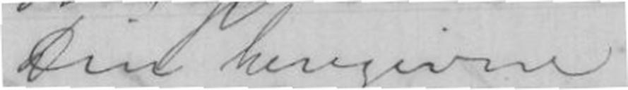Din hengivne
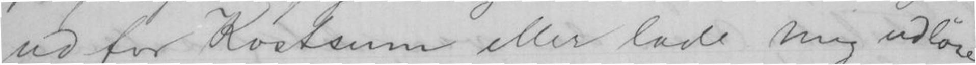ud for Kostsum eller lade mig udløse,
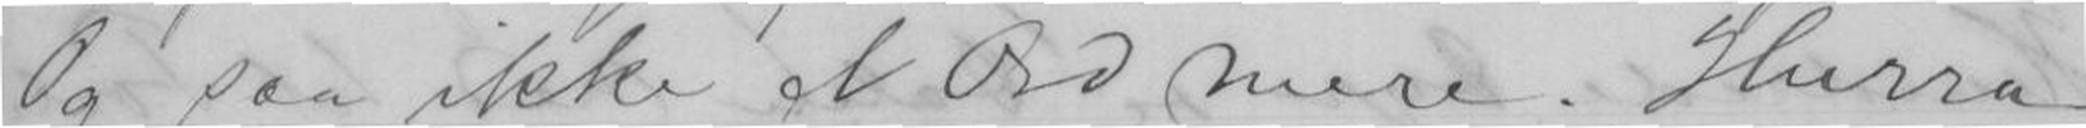Og saa ikke et Ord mere. Hurra
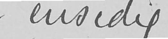ensidig
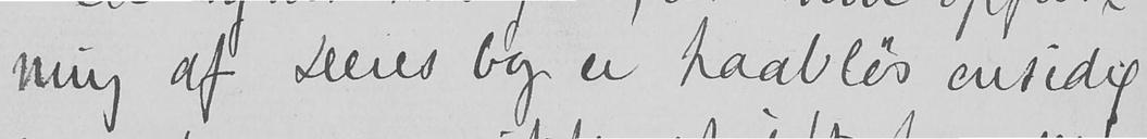ning af Deres bog er haabløs ensidig
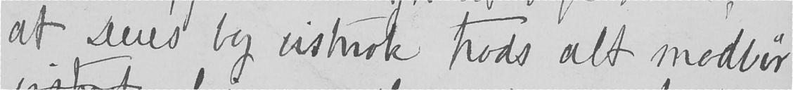at Deres bog vistnok trods alt modbør
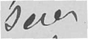saa
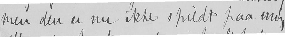men den er nu ikke spildt paa mig
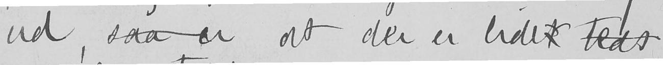ud, saa er at der er lidet teds
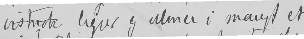vistnok ligger og ulmer i mangt et
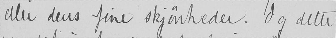eller dens fine skjønheder. Og dette
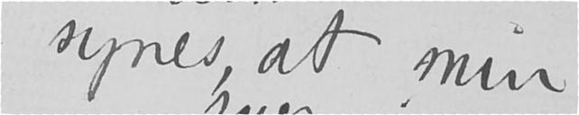synes, at min
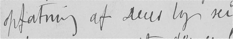opfatning af Deres bog ser
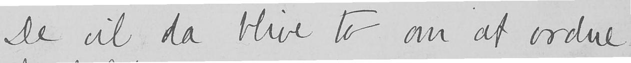De vil da blive to om at ordne
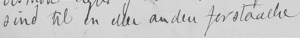sind til en eller anden forstaaelse
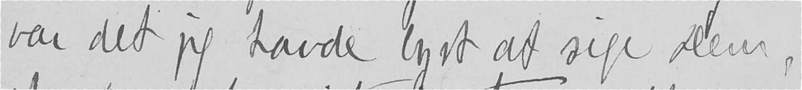var det jeg havde lyst at sige Dem,
